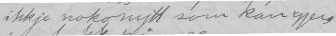ikkje noko nytt som kan gjera
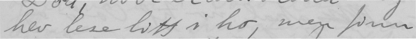hev lese litt i ho, men finn
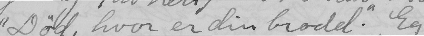"Död, hvor er din brodd." Eg
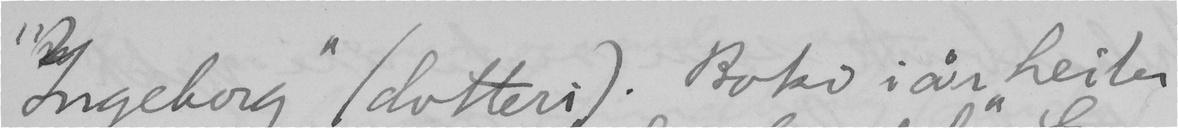"Ingeborg" (dotteri). Boki i år heiter
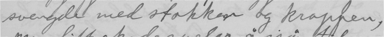svengde med stokken og kroppen,
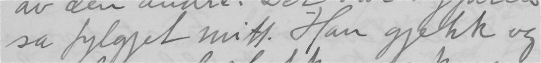sa fylgjet mitt. Han gjekk og
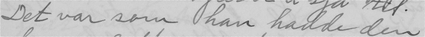Det var som han hadde den
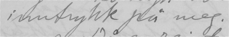inntrykk på meg.
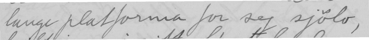lange platforma for seg sjölv,
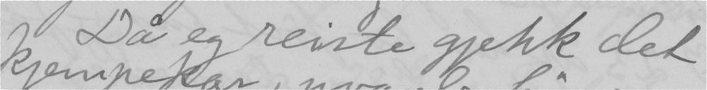Då eg reiste gjekk det
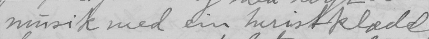musik med ein turistklædd
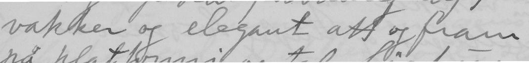vakker og elegant att og fram
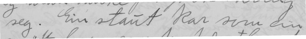seg. Ein staut kar som ein
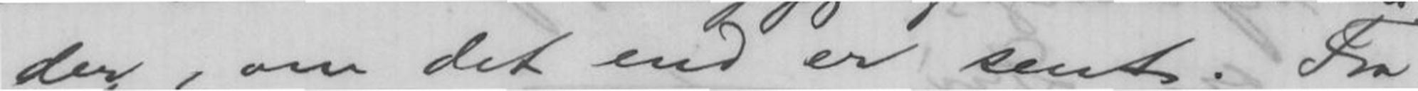der, om det end er sent. Fra
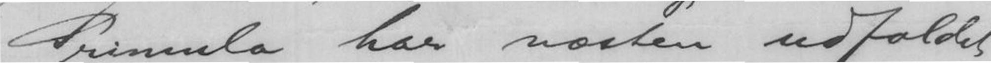Primula har næsten udfoldet
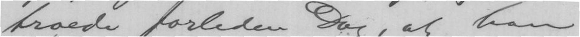troede forleden Dag, at han
